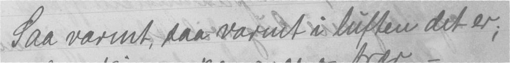Saa varmt, saa varmt i luften det er;
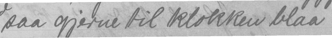saa gjerne til klokken blaa
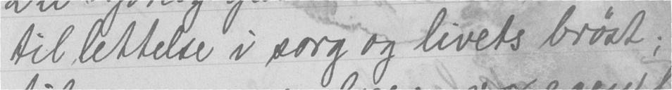til lettelse i sorg og livets brøst;
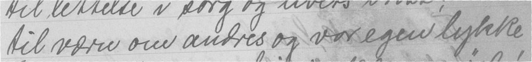til værn om andres og vor egen lykke
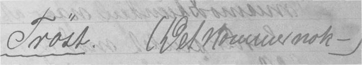Trøst. (Det kommer nok -)
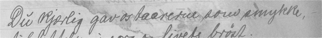Du kjærlig gav os taarerne som smykke, -
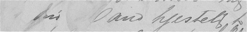i din Mand hjertelig
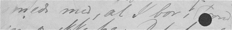mede med, at I bor i Trond-
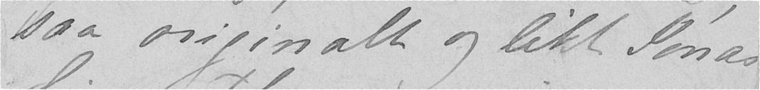saa originalt og likt Jonas
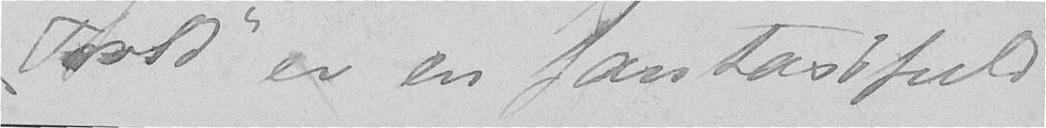Trold er en fantasifuld
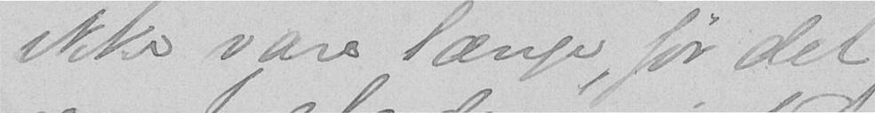ikke vare længe, før det
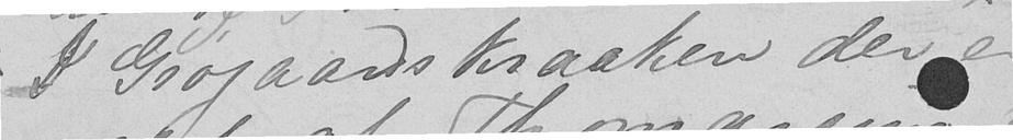I Grøgaardskraaken der er
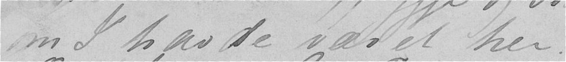om I havde været her.
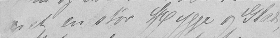været en stor Hygge og Glæde,
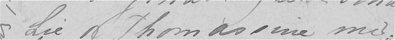Lie og Thomassine med.
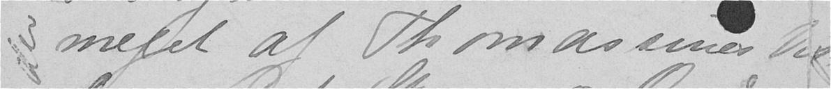meget af Thomassines Vis-
